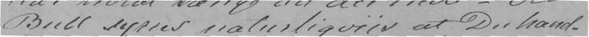Bull synes naturligviis at Du hand-
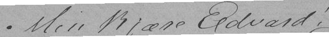Min kjære Edvard!
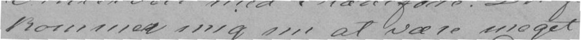kommer mig nu at være meget
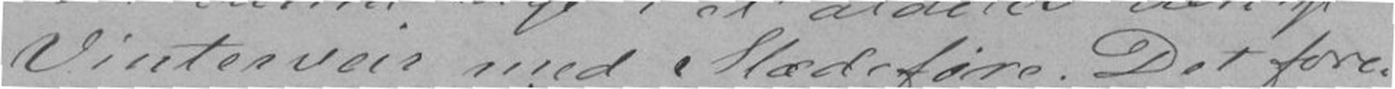Vinterveir med Slædeføre. Det fore-
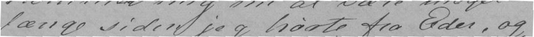længe siden jeg hørte fra Eder, og
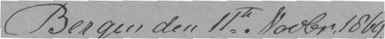Bergen den 11te Novbr. 1869.
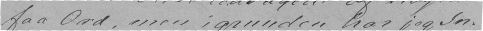faa Ord, men igrunden har jeg In-
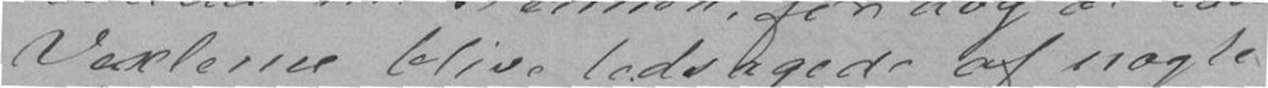Vexlerne blive ledsagede af nogle
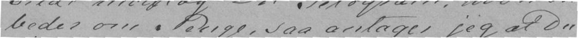beder om Penge, saa antager jeg at Du
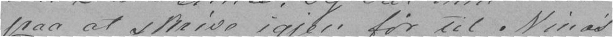paa at skrive igjen før til Ninas
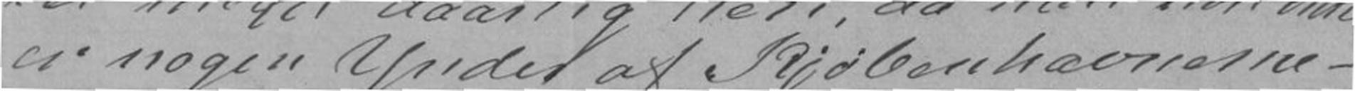er nogen Ynder af Kjøbenhavnerne -
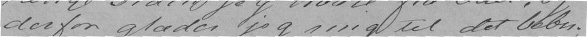derfor glæder jeg mig til det bebu-
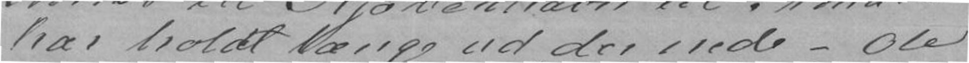har holdt længe ud der nede - Ole
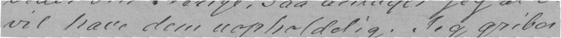vil have dem uopholddig. Jeg griber
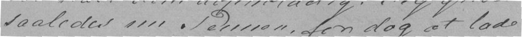saaledes nu Pennen, for dog at lade
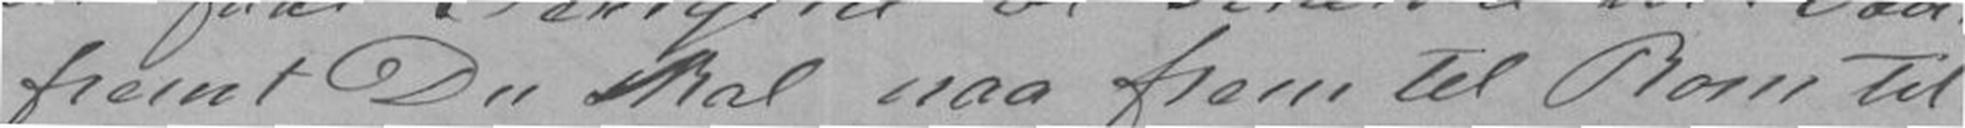fremt Du skal naa frem til Rom til
